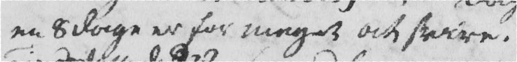en 8 Dage er for meget at skrive.
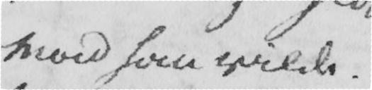Mad han vilde.
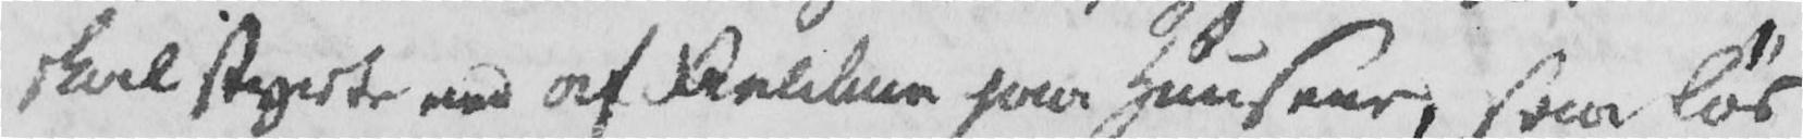skal styrte ned af Fieldene paa Huusene, saa løs
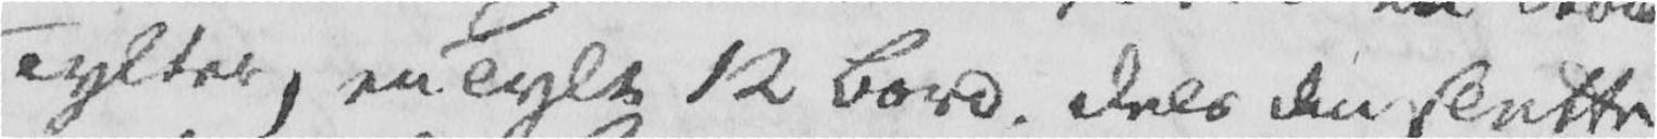Tylter, en Tylt 12 Bord. Dels den slette
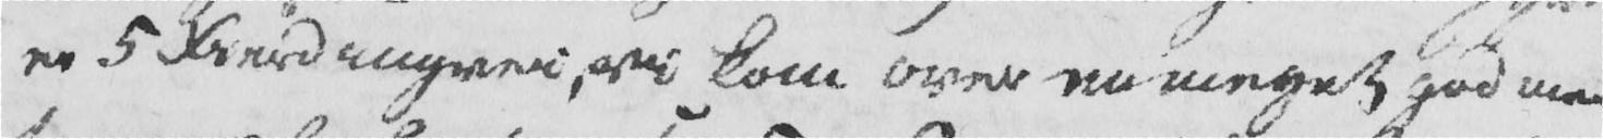er 5 Fierdingsvei, vi kom over en meget god men
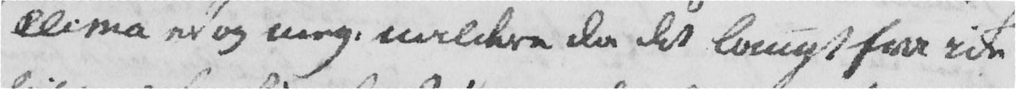Clima er og meg. mildere da det langt fra icke
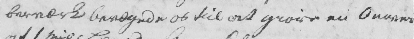berværk bevægede os til at giøre en Omvei
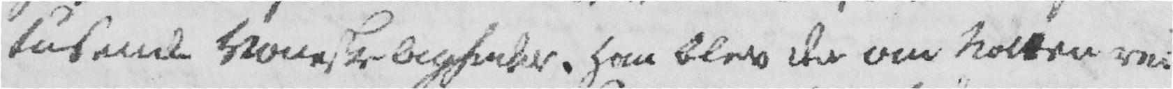tusende Vanskeligheder. Han blev der om Natten rei-
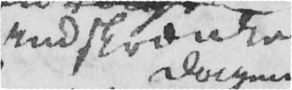indskrænke
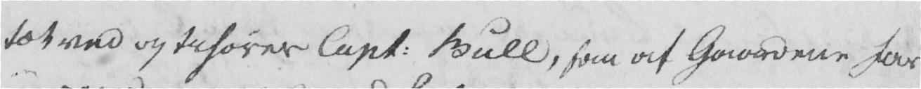tæt ved og tilhører Capt. Bull, saa at Gaardene har
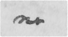er
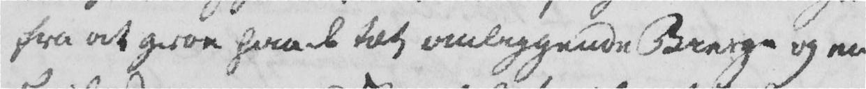fra at groe paa de tæt omliggende Bierge og en
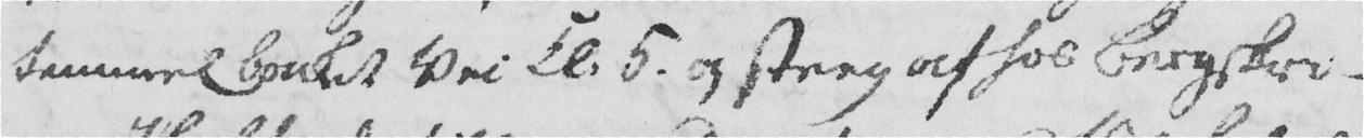temmel bonket Vei Kl. 5. og steeg af hos Bergskri-
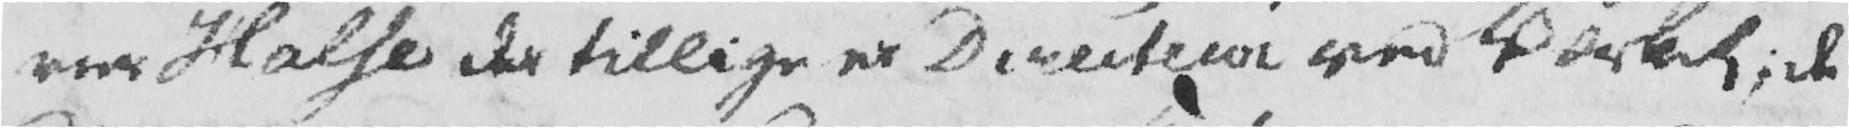ver Halse der tillige er Directeur ved Værket; de
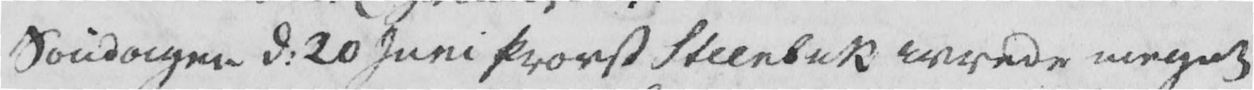Søndagen d. 20 Juni Provst Steenbuk ivrede meget
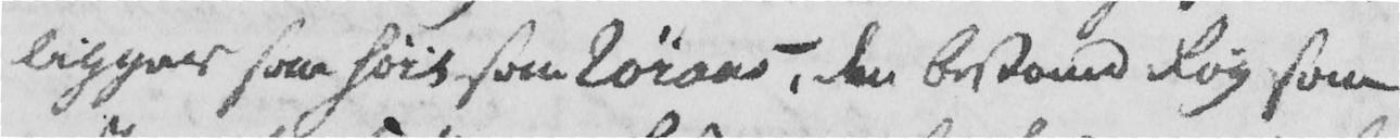ligger saa høit som Røraas, den bestand Røg som
